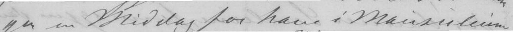ger en Middag for ham i Mausuleum
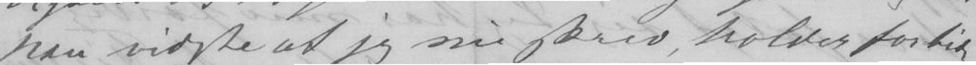han vidste at jeg nu skrev, holdes for tiden
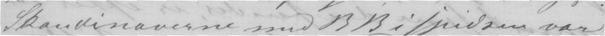Skandinaverne med B B i spidsen var
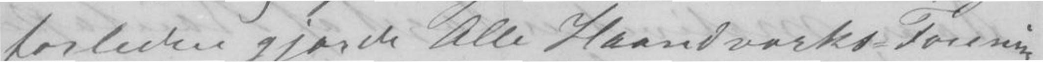forleden gjorde Alle Haandværkt=Forenin-
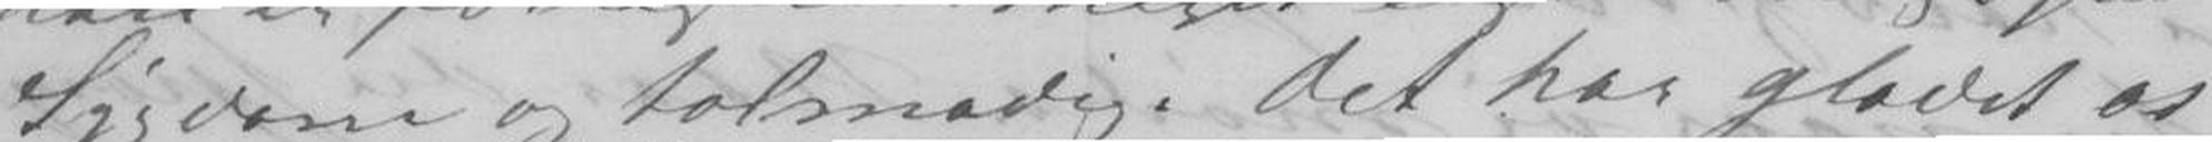Sygdom og tolmodig. Det har glædet os
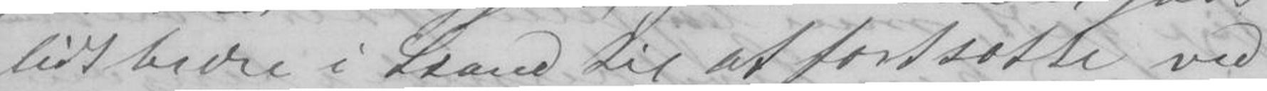lidt bedre i Stand til at fortsætte ved
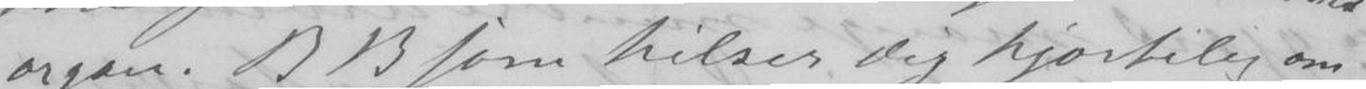organ. B B som hilser dig hjærtelig om
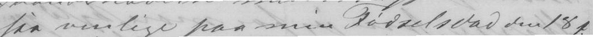saa venlige paa min Fødselsdad den 18 f.
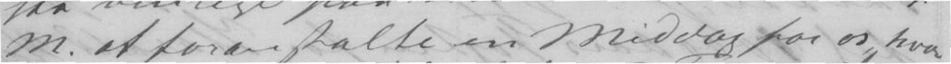M. at foranstalte en Middag for os, hva
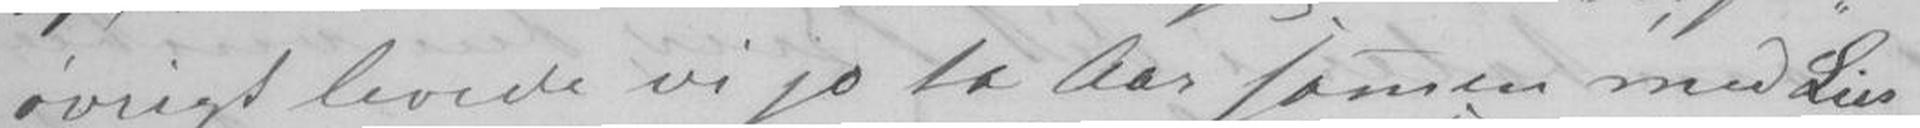øvrigt levede vi jo to Aar samen med Lies
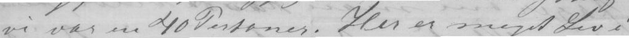vi var nu 40 Personer. Her er meget Liv i
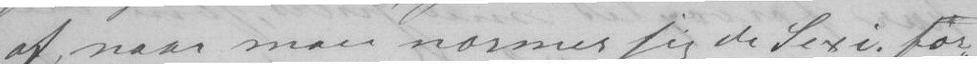af, naar man nærmer sig de Sexi; før-
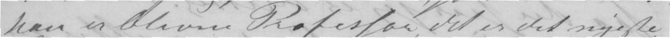han er bleven Professor, det er det nyeste
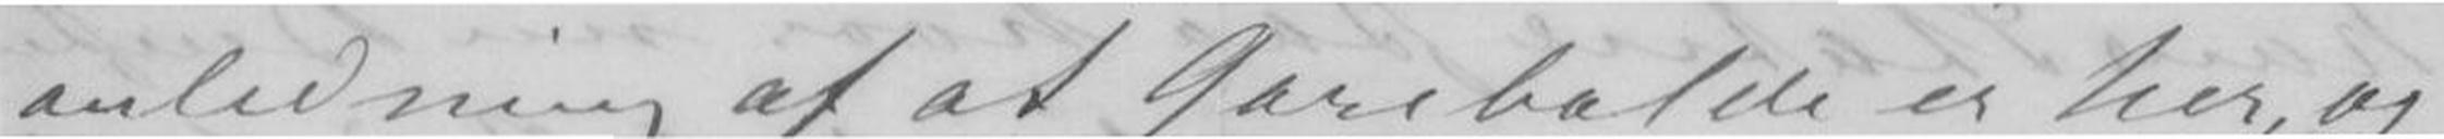anledning af at Garebalde er her, og
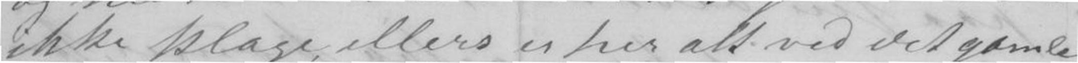ikke klage, ellers er her alt ved det gamle,
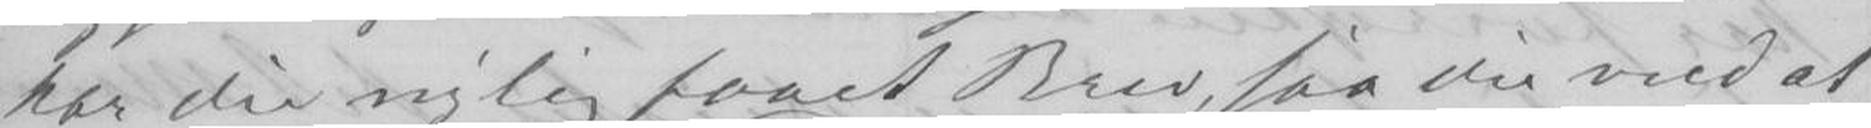har du rigtig faaet Brev, saa du veed at
